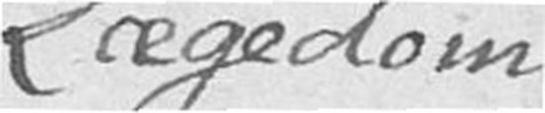Lægedom
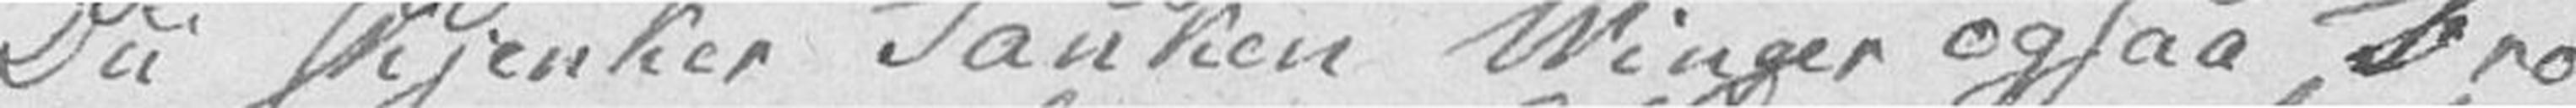Du skjenker Tanken Vinger ogsaa Tro
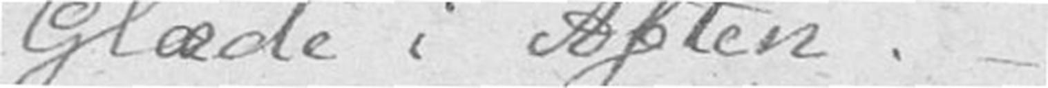Glæde i Aften. –
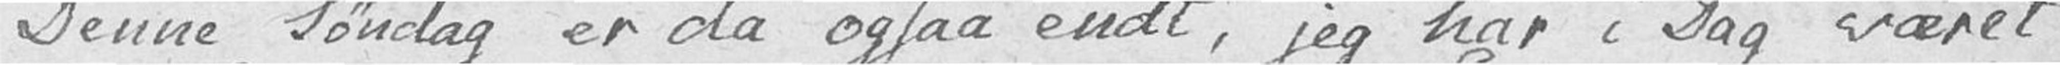Denne Søndag er da ogsaa endt, jeg har i Dag været
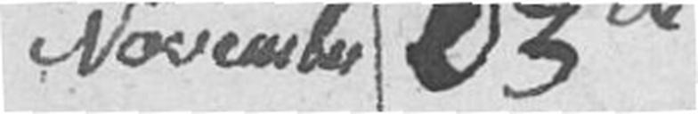November 03de
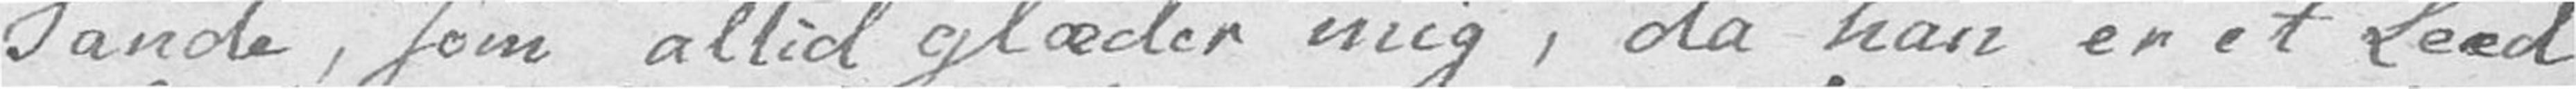Tande, som altid glæder mig, da han er et Leed
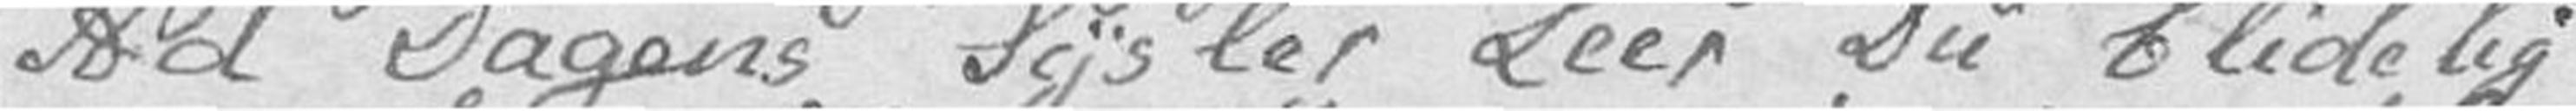Ad Dagens Sysler Leer Du blidelig
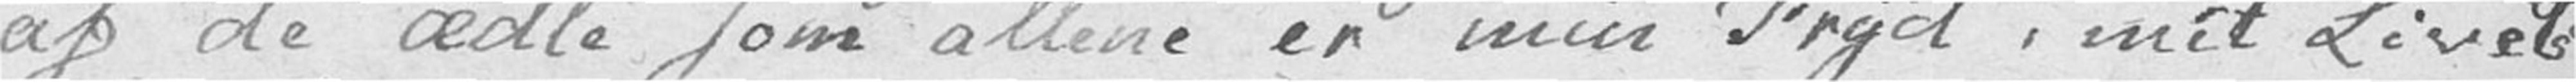af de ædle som allene er min Fryd, mit Livets
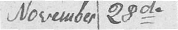November 28de
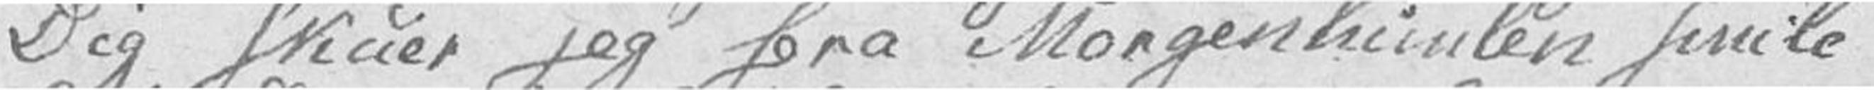Dig skuer jeg fra Morgenhimlen smile
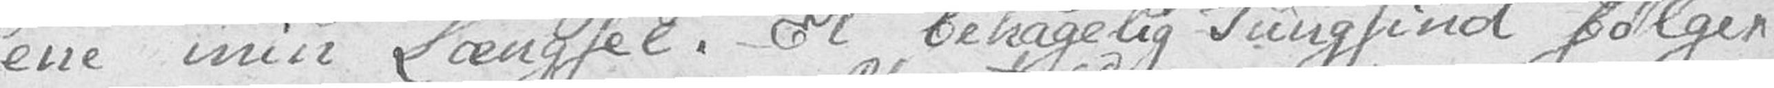ene min Længsel. Et behagelig Tungsind følger
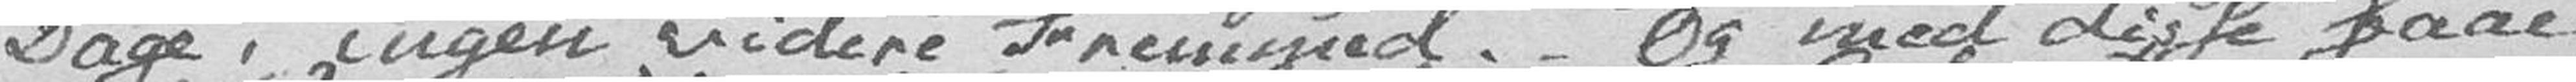Dage, ingen videre Fremmed. Og med disse faae
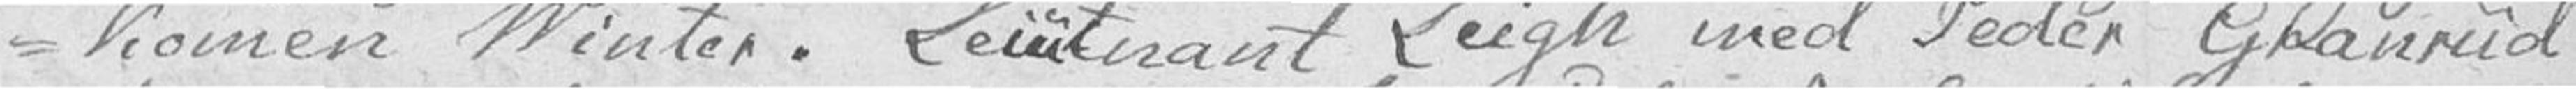-kommen Vinter. Leiutnant Leigh med Peder Granrud
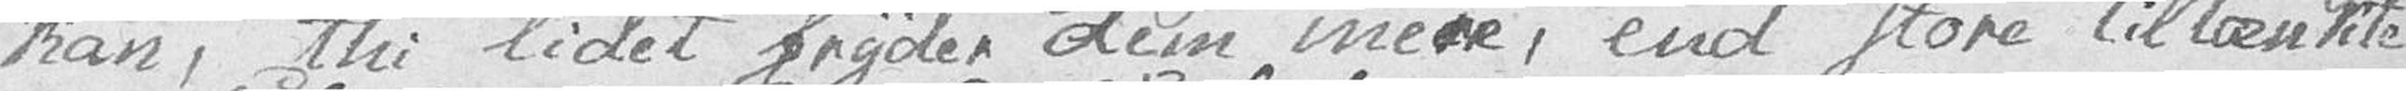kan, thi lidet fryder dem mere, end store tiltænkte
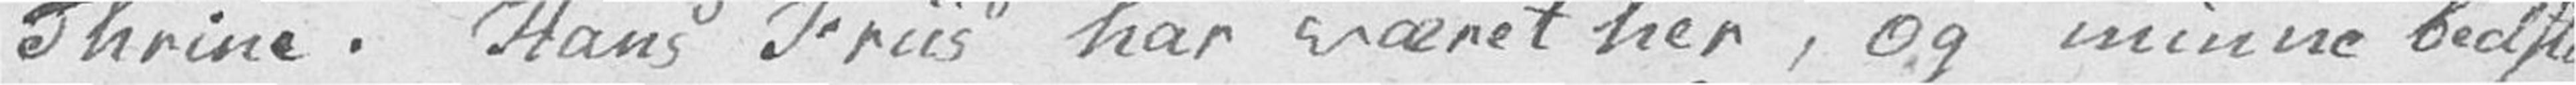Thrine. Hans Friis har været her, og minne bedste
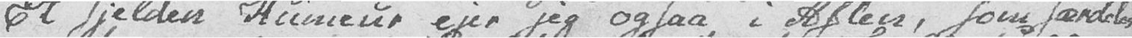Et sjelden Humeur ejer jeg ogsaa i Aften, som særdeles
